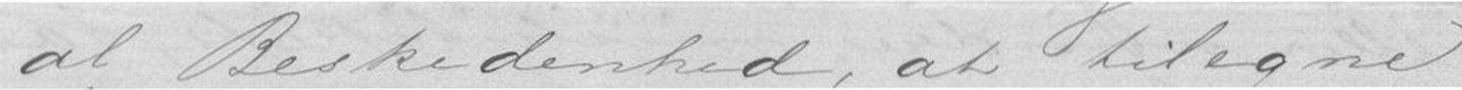al Beskedenhed, at tilegne
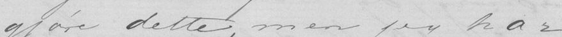gjøre dette, men jeg har
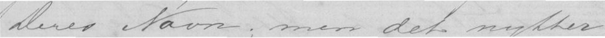Deres Navn; men det nytter
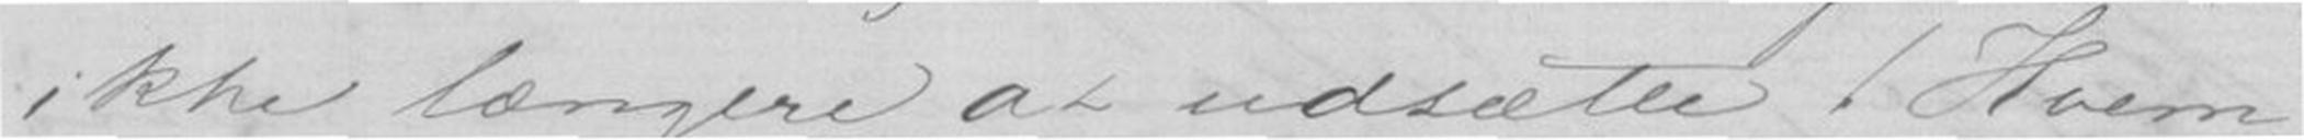ikke længere at udsætte! Hvem
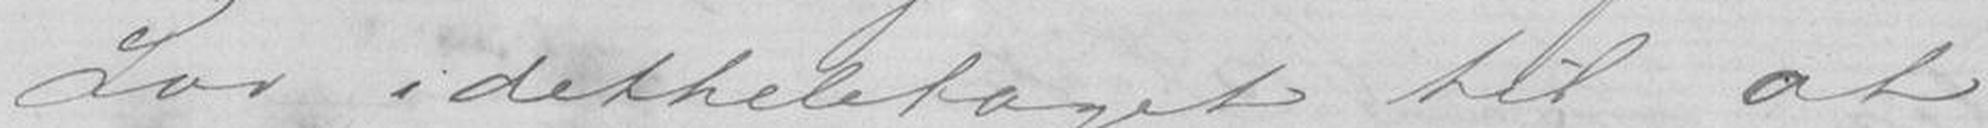Lov idetheletaget til at
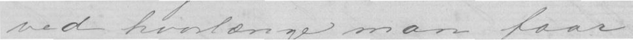ved hvorlænge man faar
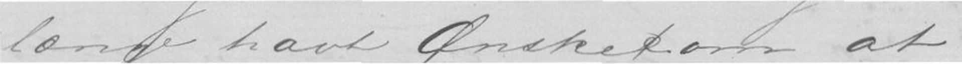lenge havt Ønsket om at
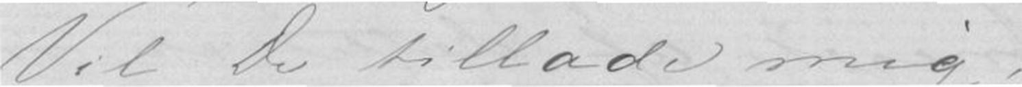Vil De tillade mig, i
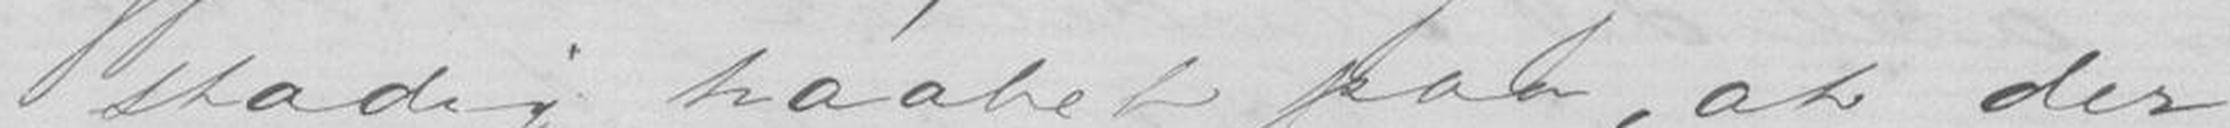stadig haabet paa, at der
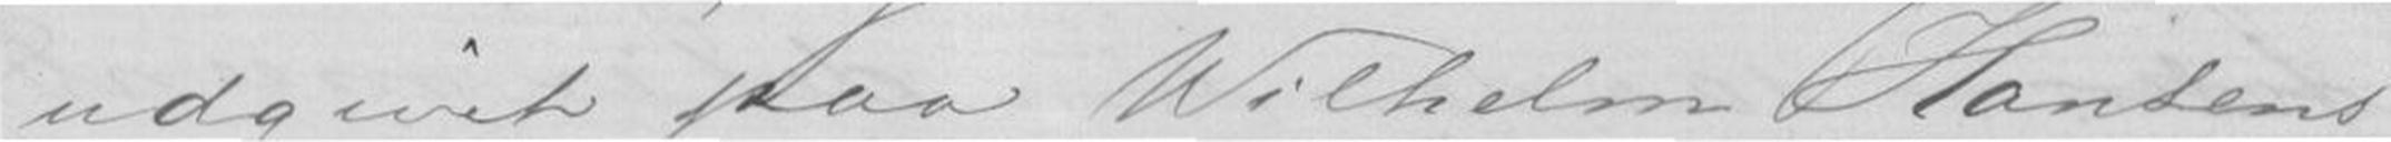utgivet paa Wilhelm Hansens
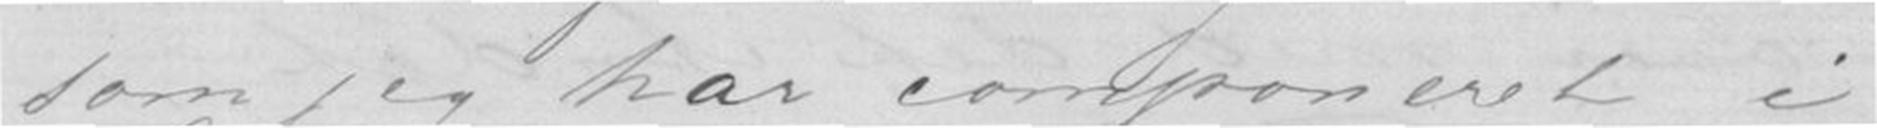som jeg har componeret i
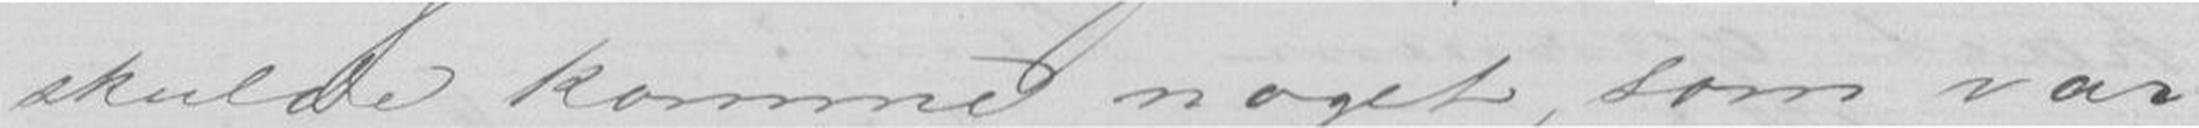skulde komme noget, som var
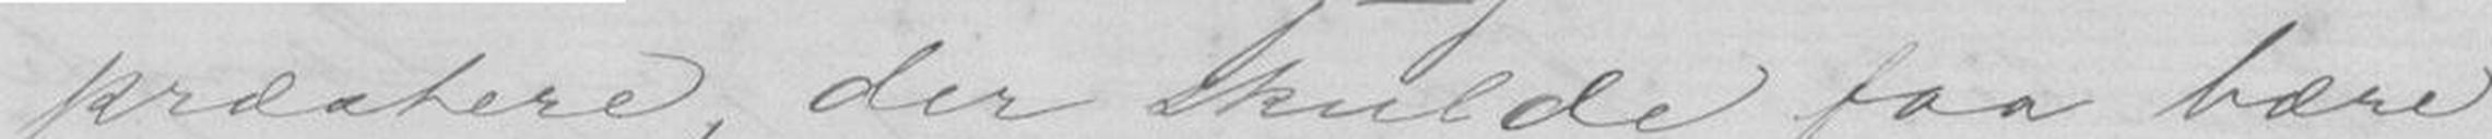præstere, der skulde faa bære
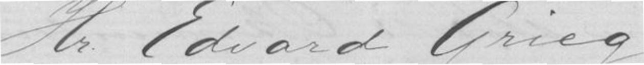Hr. Edvard Grieg!
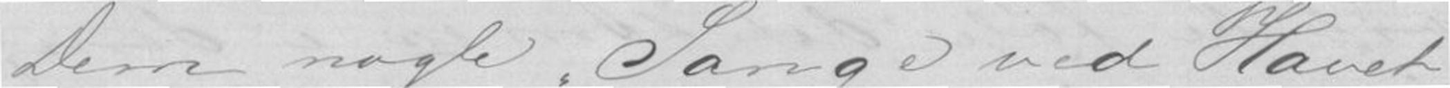Dem nogle Sange ved Havet
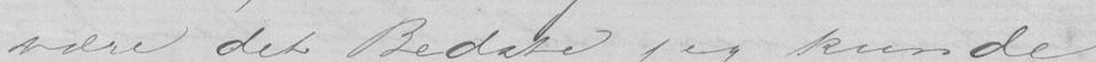være det Bedste jeg kunde
